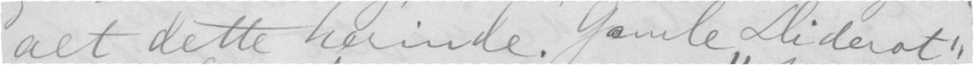alt dette herinde. Gamle Diderot
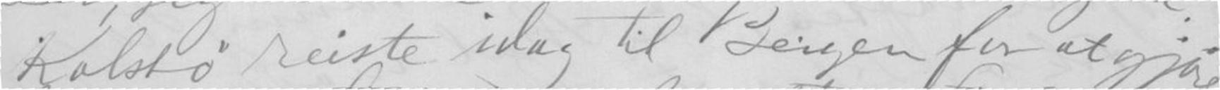Kolstø reiste idag til Bergen for at gjøre
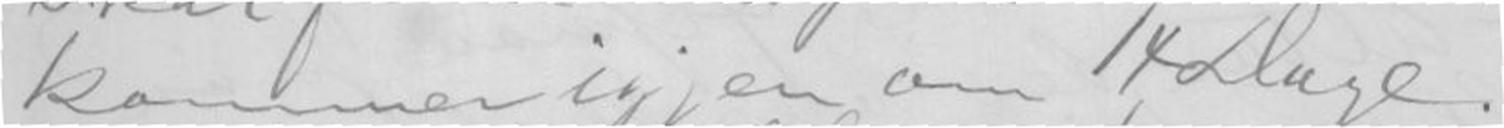kommer igjen om 14 Dage.
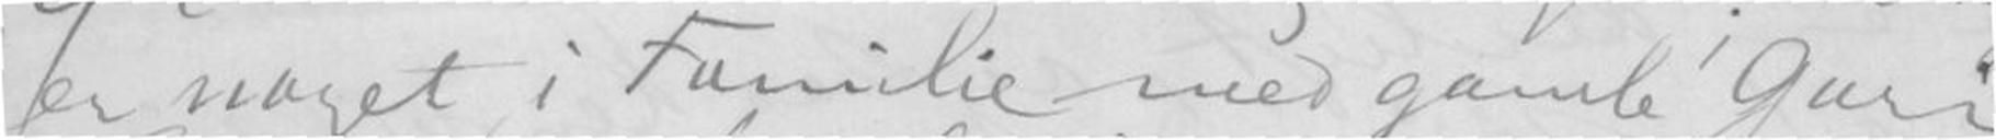er noget i Familie med gamle Guri.
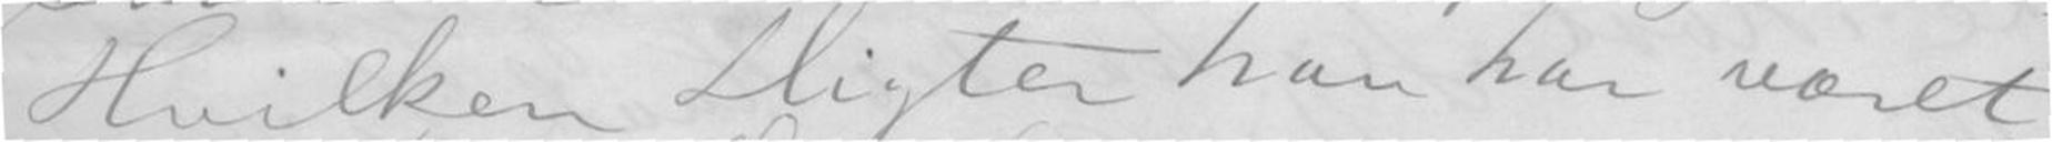Hvilken Digter han har været
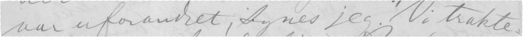var uforandret, synes jeg. Vi trakte-
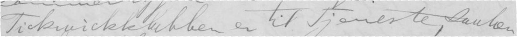Tickwickklubben er til Tjeneste, saalæn-
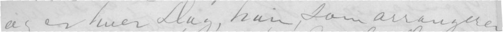og er hver Dag, han som arrangerer
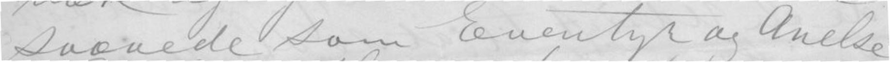svævede som Eventyr og Anelse.
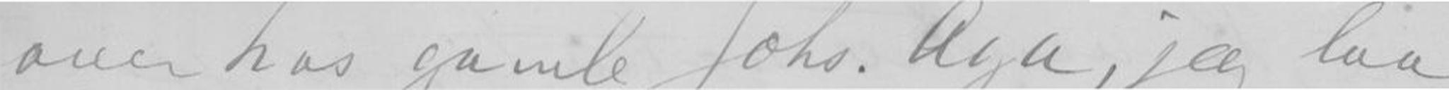over hos gamle Johs. Aga, jeg laa
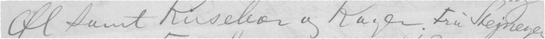Øl samt Kirseebær og Kager; Fru Steineger
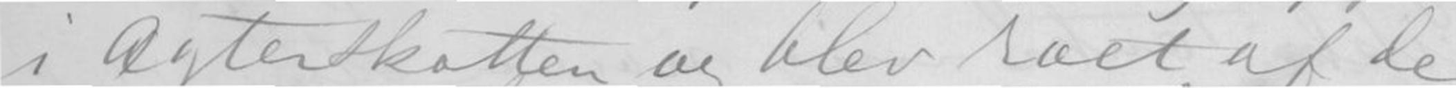i Agterskotten og blev roet af de
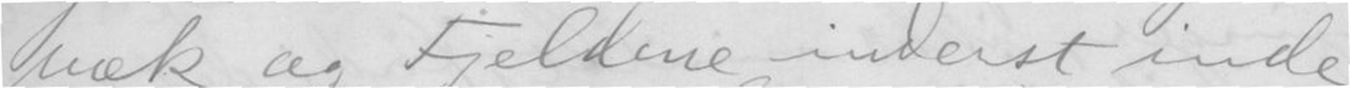væk og Fjældene inderst inde
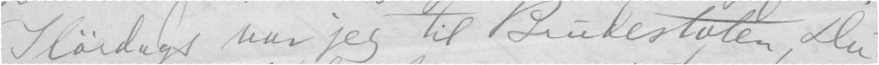Ilørdags var jeg til Brudestolen, Du
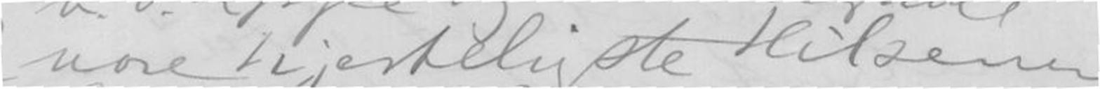vore hjerteligste Hilsener
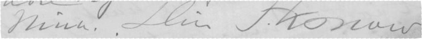og Nina. Din F. Konow
